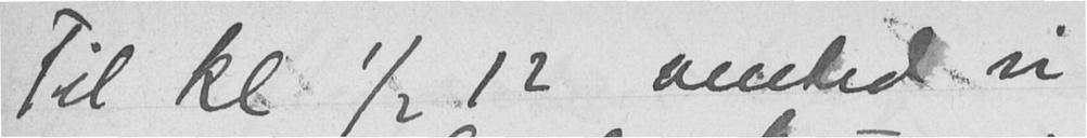Til kl. ½ 12 vented vi
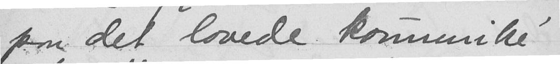paa det lovede komuniké
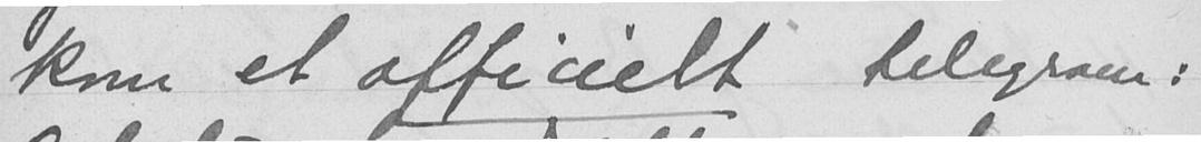kom et officielt telegram:
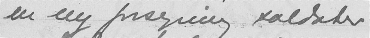en ny forsyning soldater
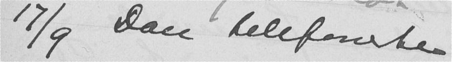17/9 Don telefonerte
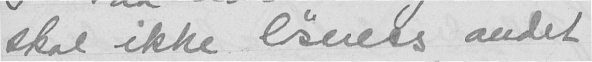skal ikke løsenes andet
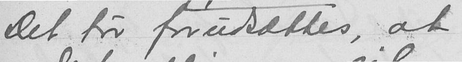Det tør forudsættes, at
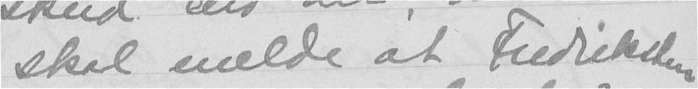skal melde at Fredriksten
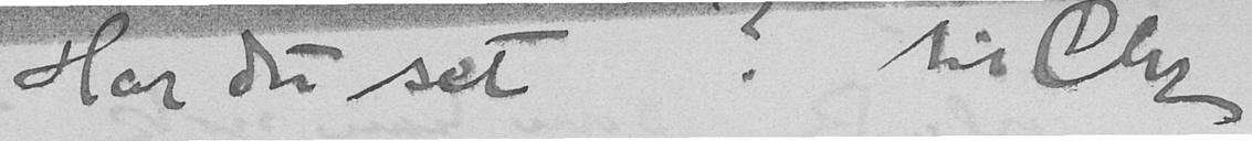Har du set? til Chr.
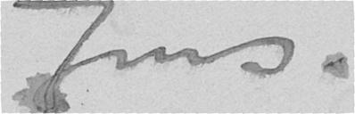Jens.
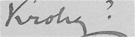Krohg?
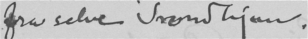fra selve Trondhjem.
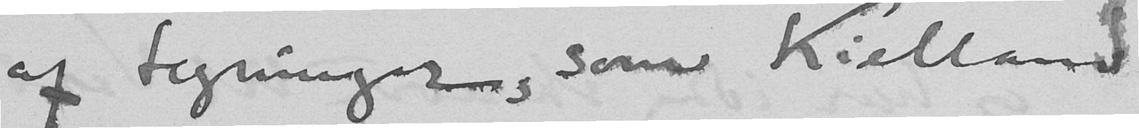af tegninger, som Kielland
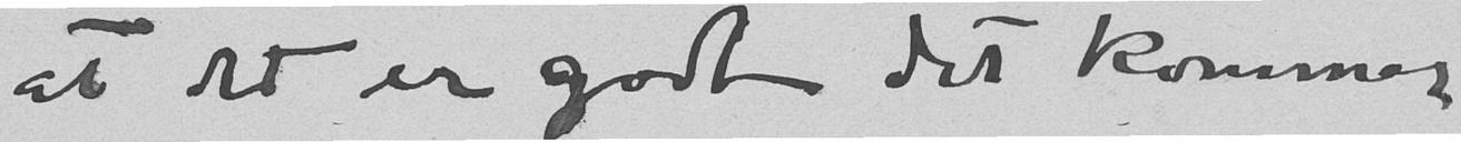at det er godt det kommer
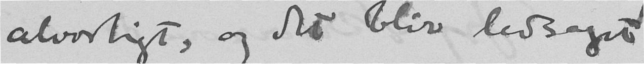alvorligt, og det blir ledsaget
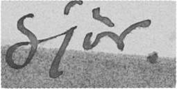gjør.
